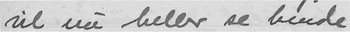vil nu heller se hende
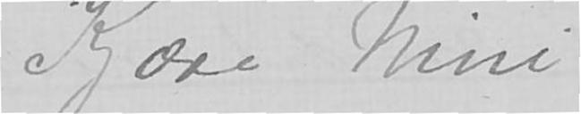Kjære Nini
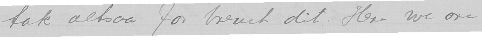tak altsaa for brevet dit. Here we are
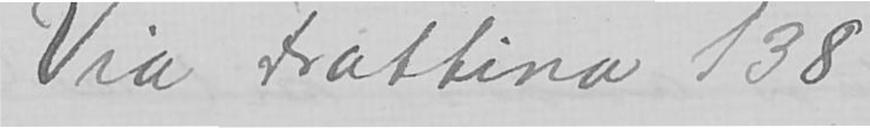Via Fratina 138
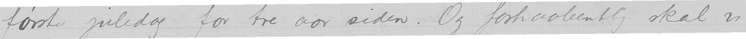første juledag for tre aar siden. Og forhaabentlig skal vi
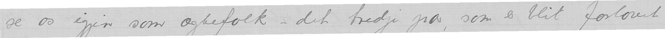se os igjen som ægtefolk - det tredje par, som er blit forlovet
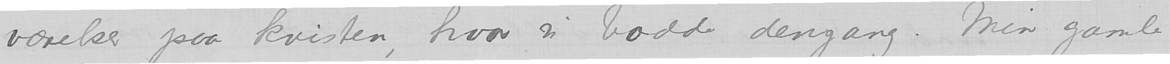værelser paa kvisten, hvor vi bodde dengang. Min gamle
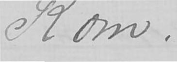Rom.
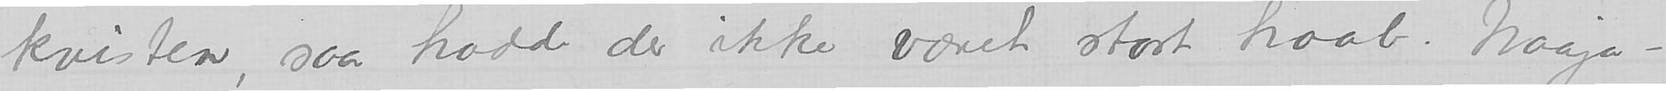kvisten, saa hadde der ikke været stort haab. Naaja-
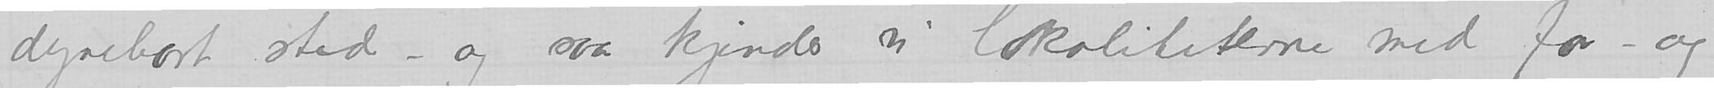et dyrebart sted – og saa kjender vi lokaliteterne med for- og
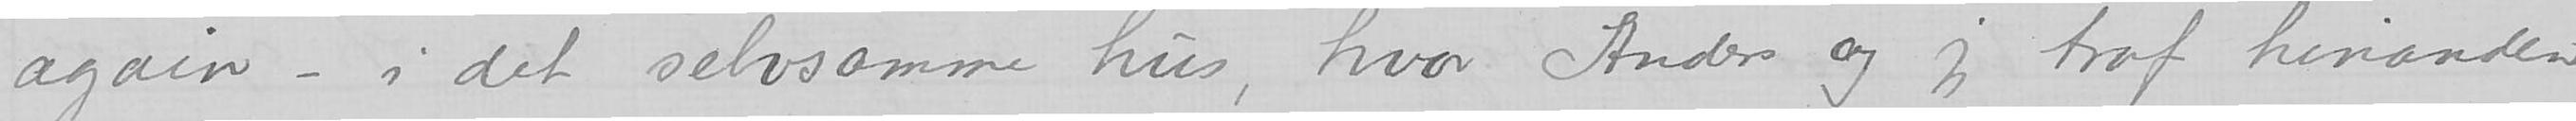again – i det selvsamme hus, hvor Anders og jeg traf hinanden
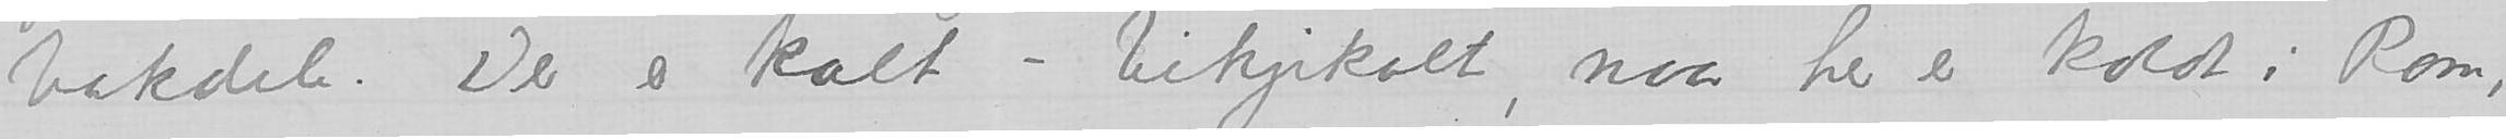bakdele. Der er kalt - bikjekalt, naar her er koldt i Rom,
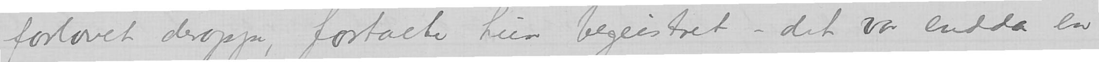par forlovet deroppe, fortalte hun begeistret – det var endda en
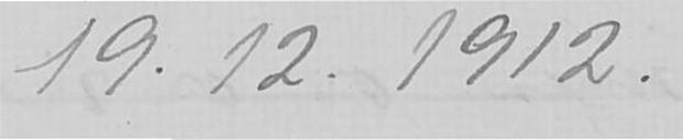19.12.1912
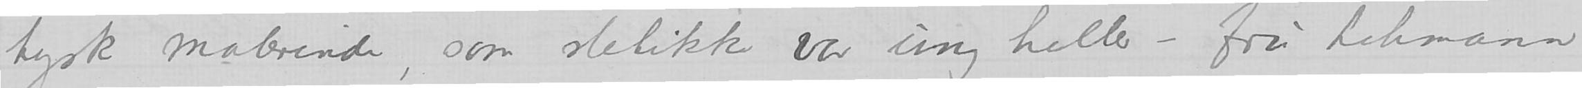tysk malerinde, som sletikke var ung heller – fru Lehmann
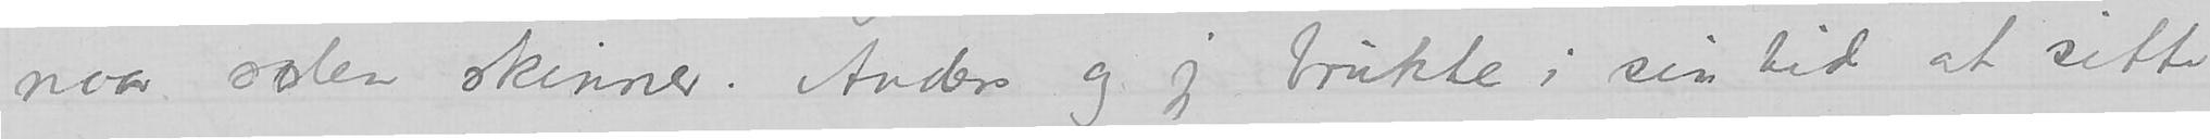naar solen skinner. Anders og jeg brukte i sin tid at sitte
